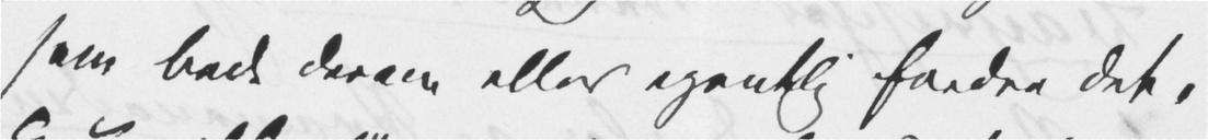som bede derom eller egentlig fordre det,
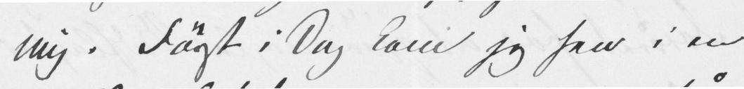mig. Først i Dag kom jeg hen i en
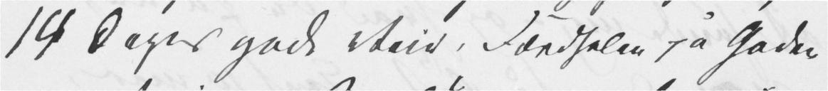14 Dages godt Veir, Færdselen på Gaden
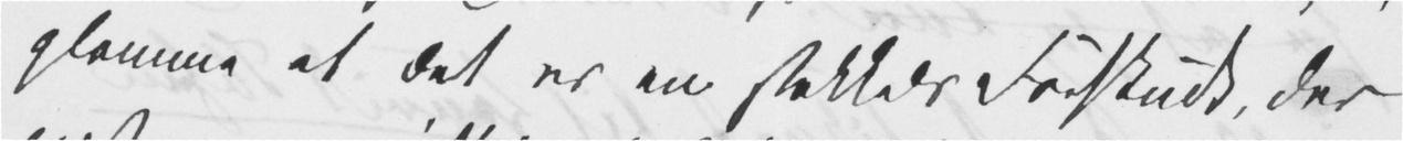glemme at det er en stakkels Forskudt, der
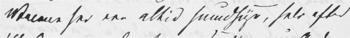Veiene her ere altid smudsige, selv efter
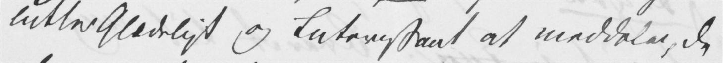lutter Glædeligt og Interessant at meddele, de
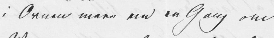i Ovnen mere end en Gang om
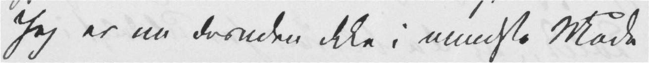Jeg er nu desuden ikke i mindste Måde
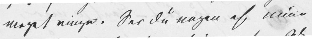meget ringe. Ser Du nogen af mine
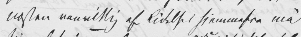næsten vanvittig af Lidelser hjemmefra må
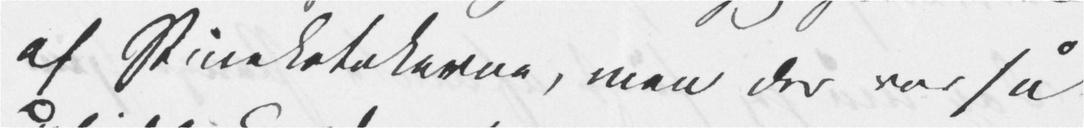af Pinekatakerne, men der var så
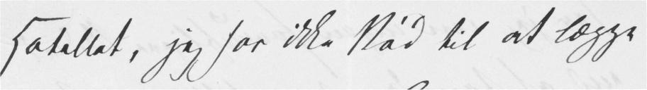Hotellet, jeg har ikke Råd til at lægge
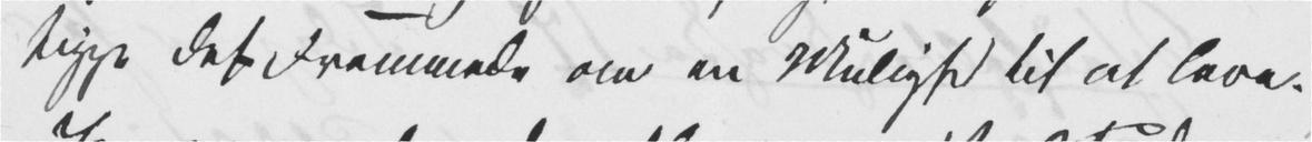tigge det Fremmede om en Mulighed til at leve.
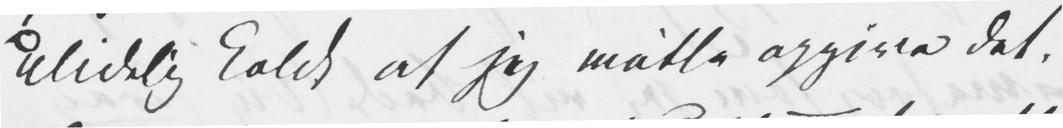ulidelig koldt at jeg måtte opgive det.
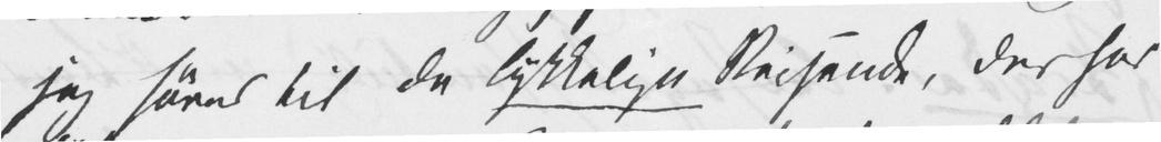jeg hører til de lykkelige Reisende, der har
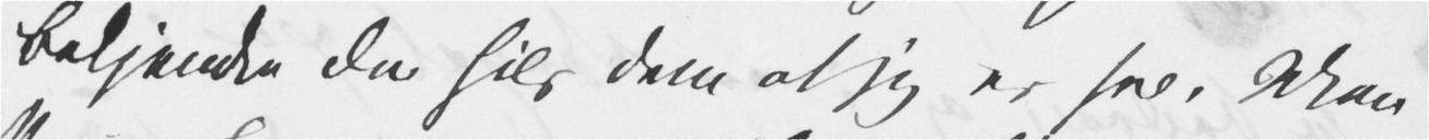Bekjendte da hils dem at jeg er her, Men
